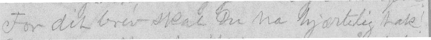For dit brev skal Du ha hjærtelige tak!
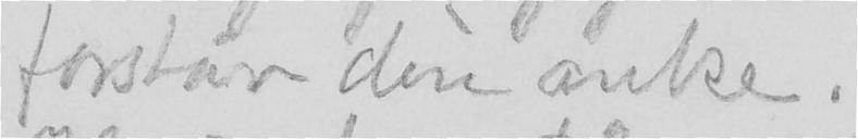forstår dèn anke.
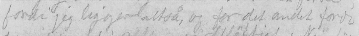fordi jeg ligger altså, og for det andet fordi
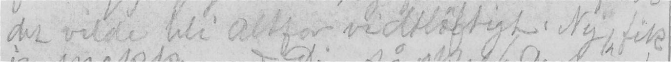det vilde bli altfor vidtløftigt. Nej, fik
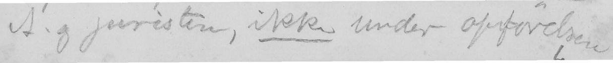A. og juristen, ikke under opførelsen
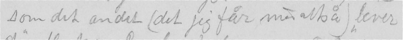som det andet (det jeg får med altså) "lever
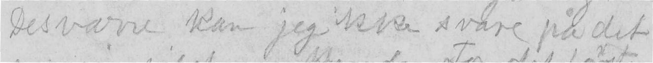Desværre kan jeg ikke svare på det
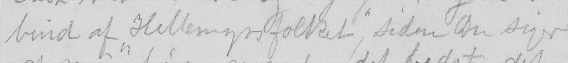bind af "Hellemyrsfolket", siden Du siger
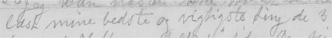læst mine bedste og vigtigste ting de 3
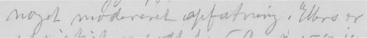noget modereret opfatning. Ellers er
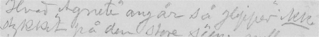Hvad "Agnete" angår så "glipper" ikke
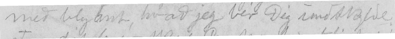med blyant hvad jeg bér Dig undskylde.
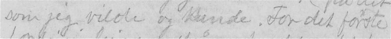som jeg vilde og kunde. For det første
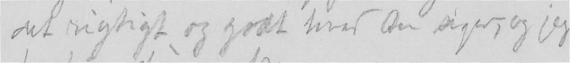det rigtigt, og godt hvad Du siger, og jeg
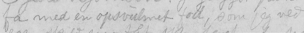fa med en opsvulmet fod, som jeg ved
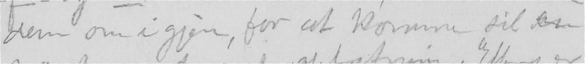dem om igjen, for at komme til en
